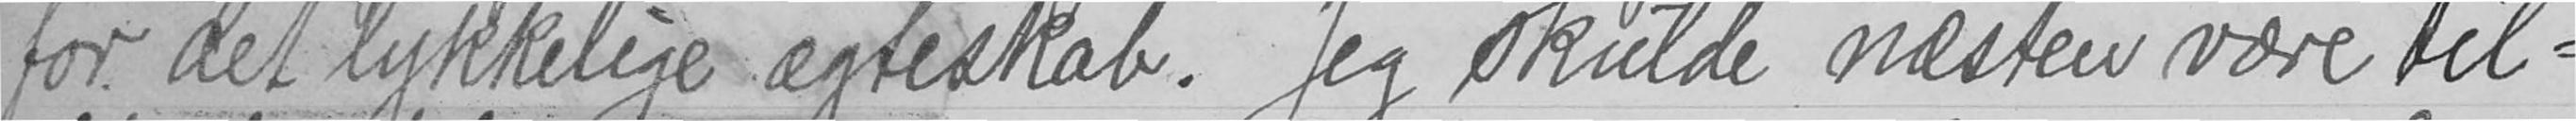for det lykkelige ægteskab. Jeg skulde næsten være til-
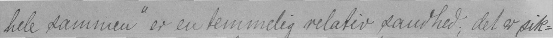hele sammen" er en temmelig relativ sandhed; det er sik-
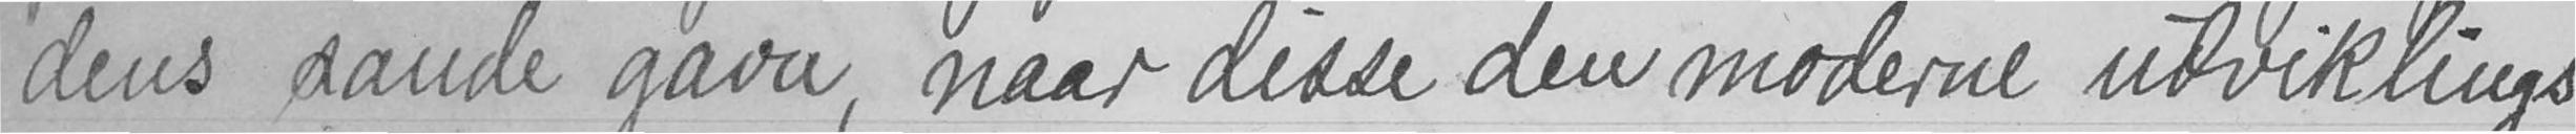dens sande gavn, naar disse den moderne udviklings
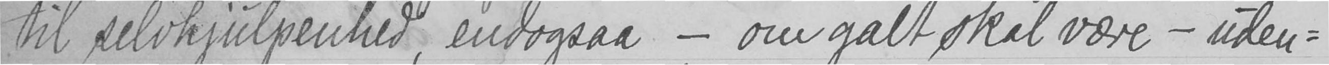til selvhjulpenhed, endogsaa - om galt skal være - uden-
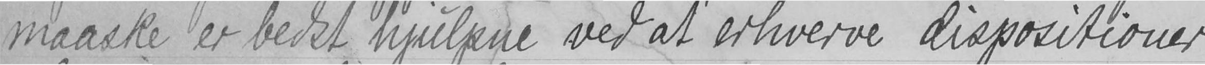maaske er bedst hjulpne ved at erhverve dispositioner
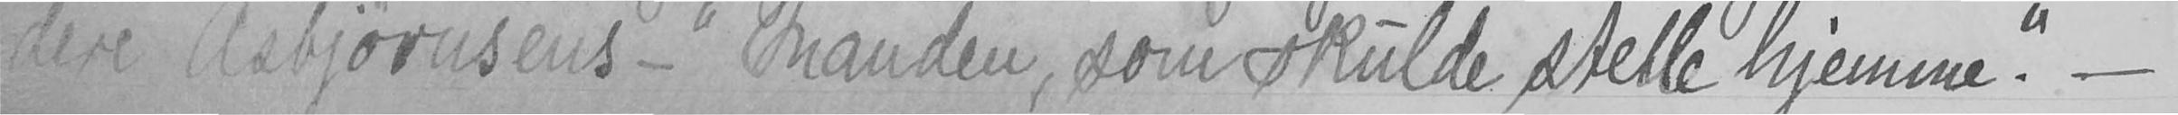dere Asbjørnsens - "Manden, som skulde stelle hjemme." -
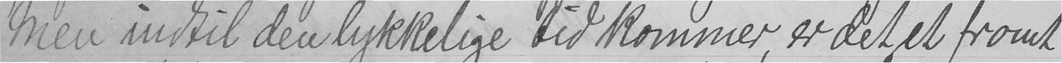men indtil den lykkelige tid kommer, er det et fromt
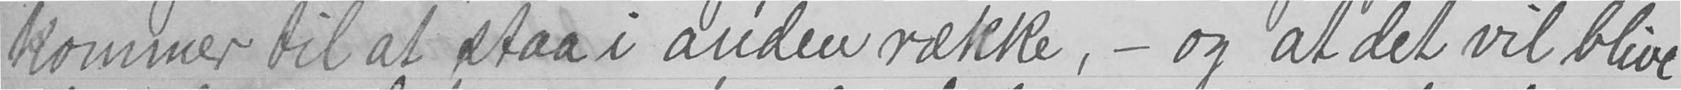kommer til at staa i anden række, - og at det vil blive
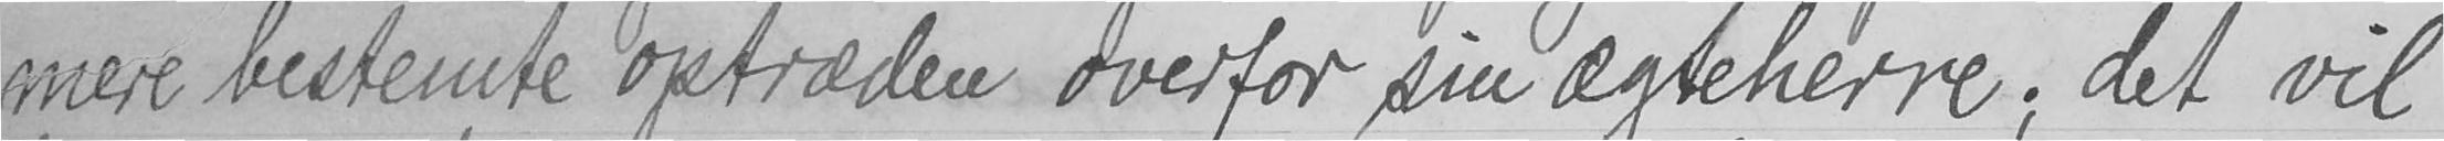mere bestemte optræden overfor sin ægteherre; det vil
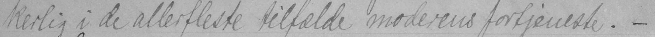kerlig i de allerfleste tilfælde moderens fortjeneste. -
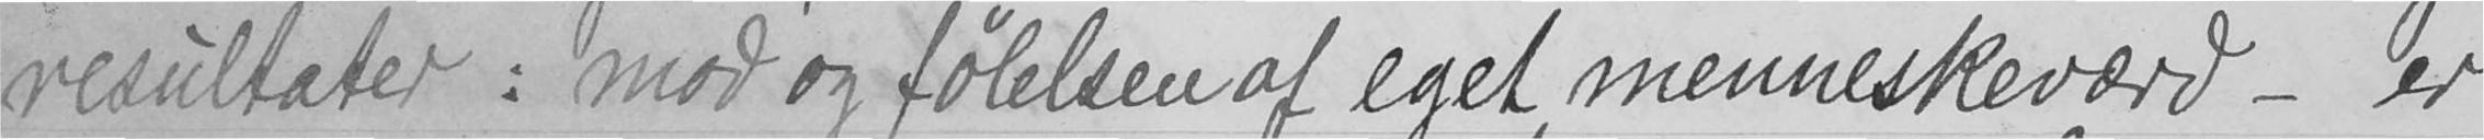resultater: mod og følelsen af eget menneskeværd - er
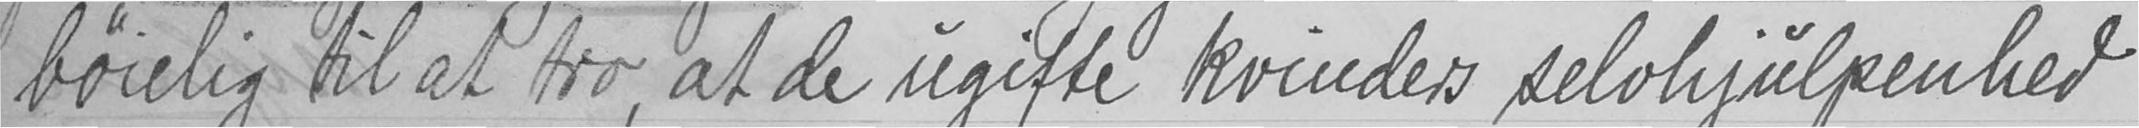bøielig til at tro, at de ugifte kvinders selvhjulpenhed
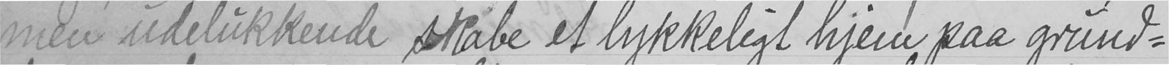men udelukkende skabe et lykkeligt hjem paa grund-
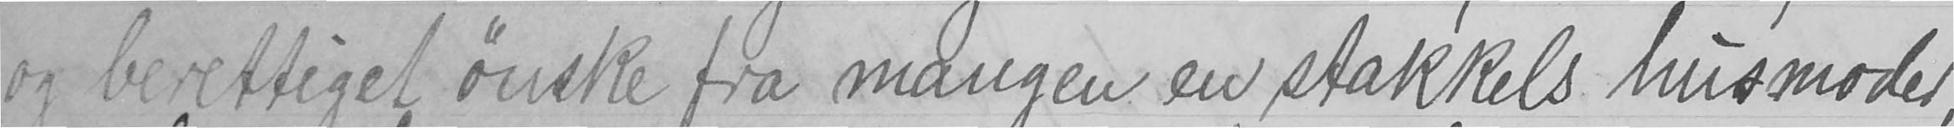og berettiget ønske fra mangen en stakkels husmoder,
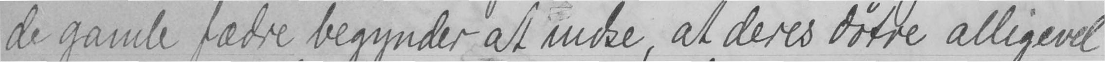de gamle fædre begynder at indse, at deres døtre alligevel
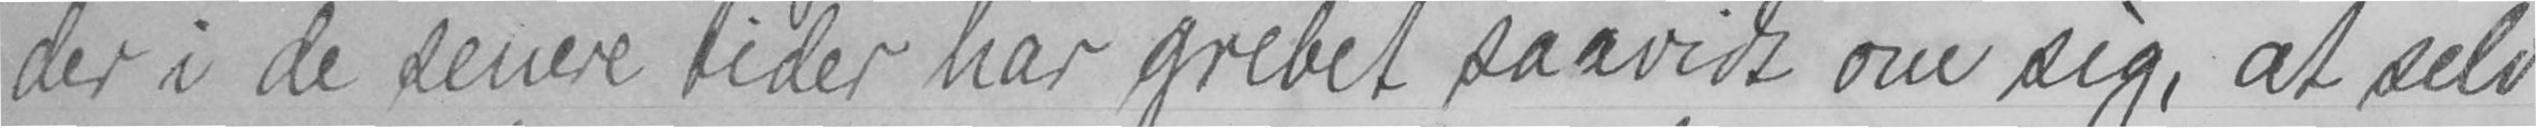der i de senere tider har grebet saavidt om sig, at selv
